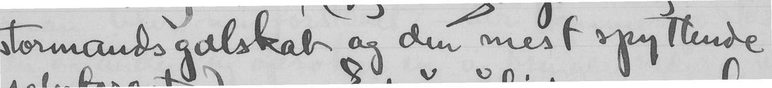stormands galskab og den mest spyttende
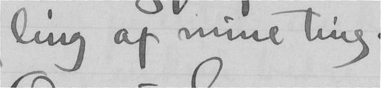ling af mine ting.
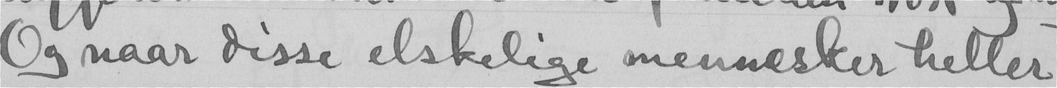Og naar disse elskelige mennesker heller
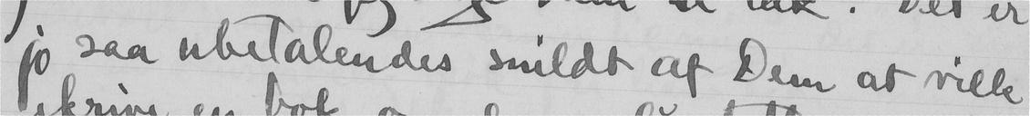jo saa ubetalendes snildt af Dem at ville
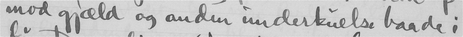mod gjæld og anden underkuelse baade i
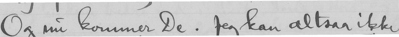Og nu kommer De. Jeg kan altsaa ikke
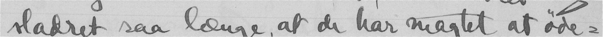sladret saa længe, at de har magtet at øde-
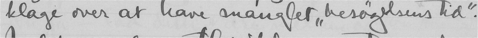klage over at have manglet "besøgelsens tid".
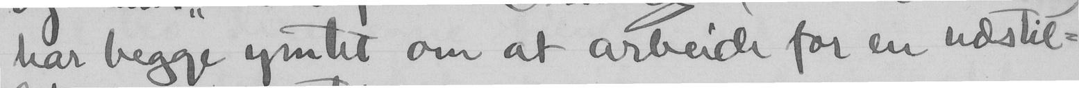har begge ymtet om at arbeide for en udstil-
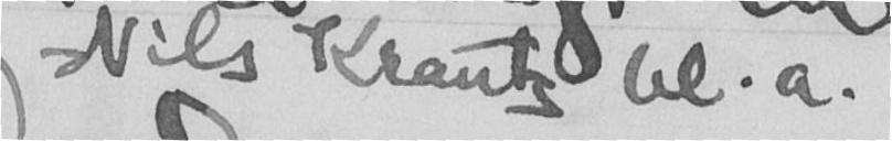Nils Krantz bl.a.
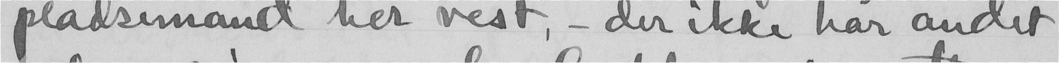pladsemand her vest, - der ikke har andet
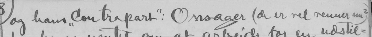Dog hans "contrapart": Onsager (de er vel venner nu?)
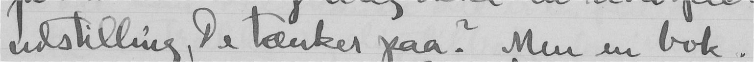udstilling, De tænker paa? Men en bok.
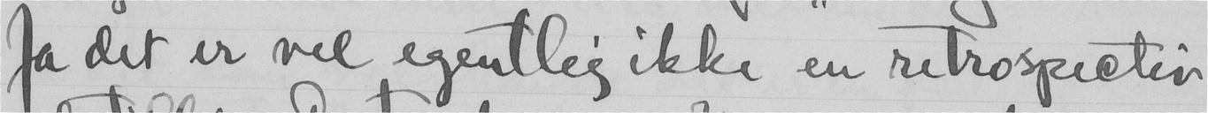Ja det er vel egentlig ikke en retrospectiv
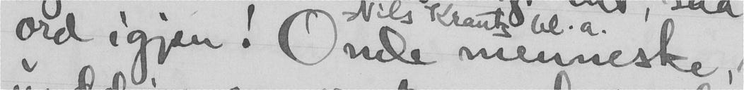ord igjen. Onde menneske,
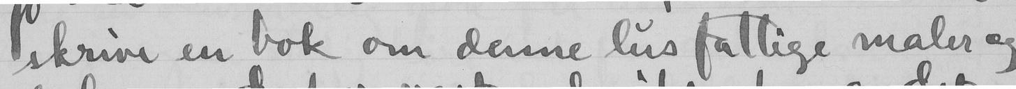skrive en bok om denne lusfattige maler og
